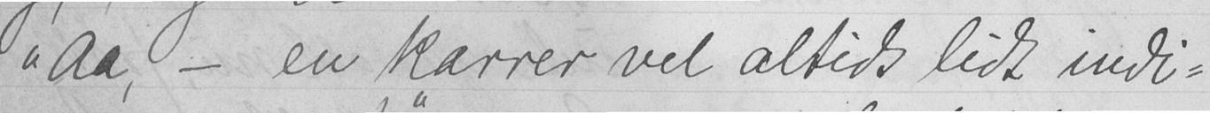"Aa, - en karrer vel altids lidt indi-
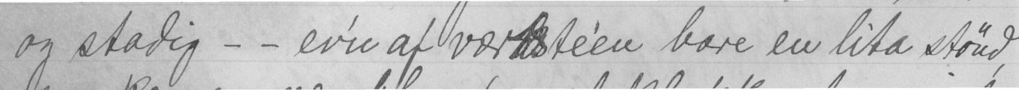og stadig - - er'n af værdste'en bare en lita stønd,
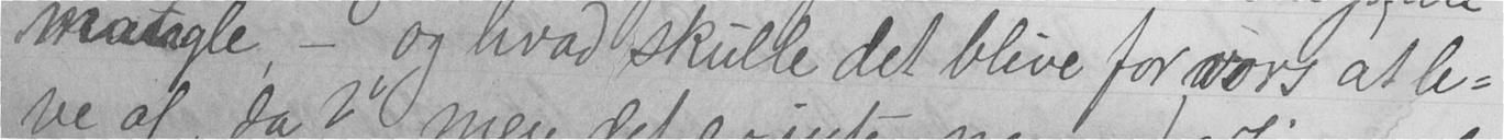mangle, - og hvad skulle det blive for vors at le-
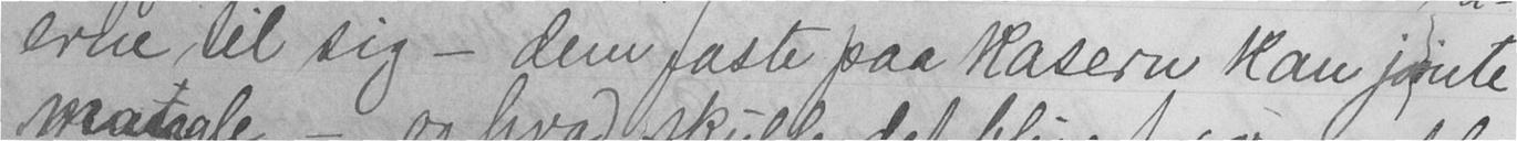erne til sig - dem faste paa kasern kan jo inte
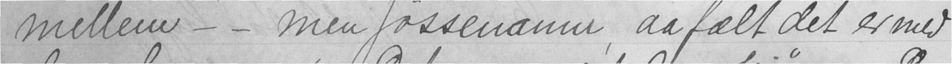mellem - - men Jøssenamm, aa fælt det er med
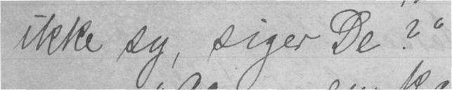ikke sy, siger De?"
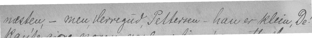næsten, - men, Herregud, Pettersen - han er klein, De!
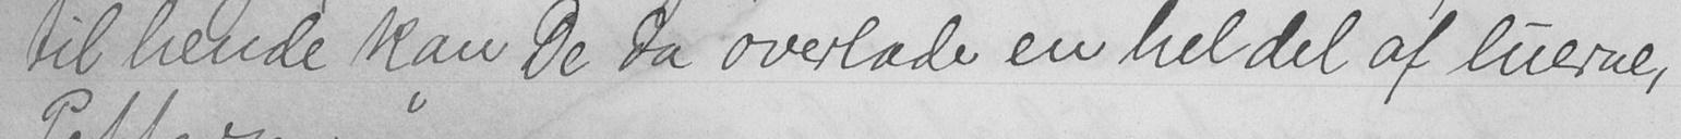til hende kan De da overlade en hel del af luerne,
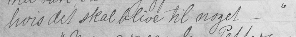hvis det skal blive til noget - "
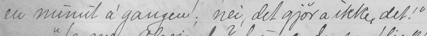en minut a' gangen; nei, det gjør a ikke, det!"
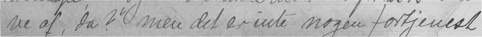ve af, da?" men det er inte nogen fortjenest
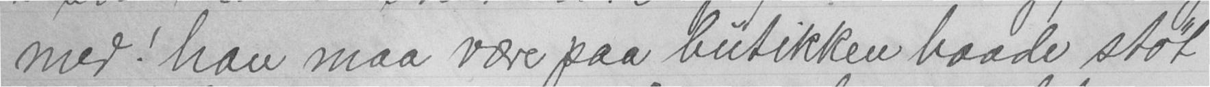med! han maa være paa butikken baade støt
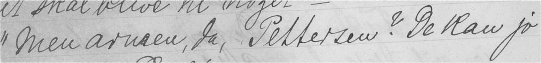"Men armen, da, Pettersen? De kan jo
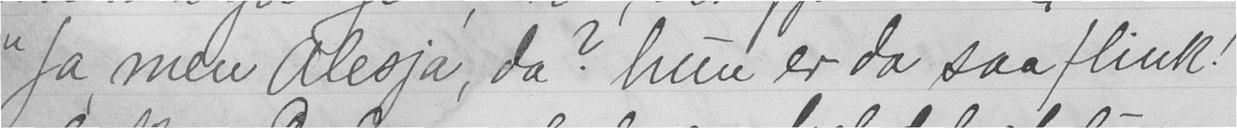"Ja, men Alesja, da? hun er da saa flink!
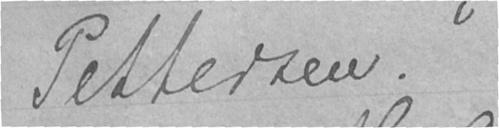Pettersen. "
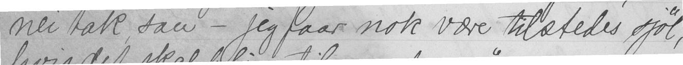nei tak, san - jeg faar nok være tilstedes sjøl,
